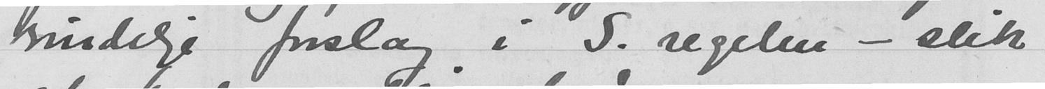rindelige forslag i 5. regelen - slik
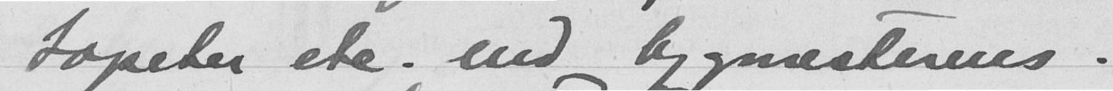tapeter etc. end bygmesterens.
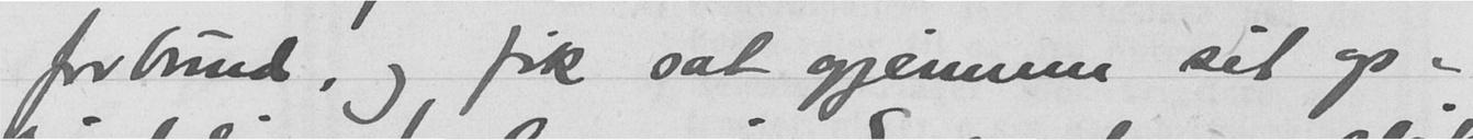forbund, og fik sat gjennem sit op-
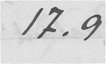17.9
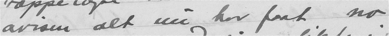avisen alt nu har faat no
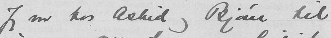Jeg var hos Astrid og Bjørn til
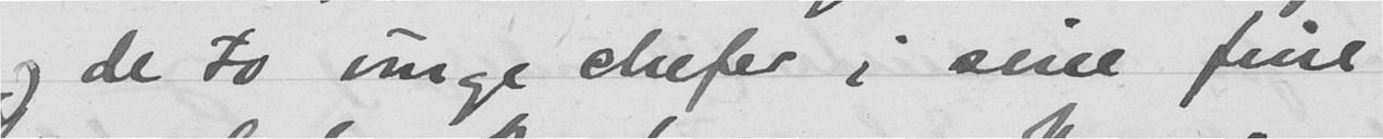og de to unge chefer i sine fine
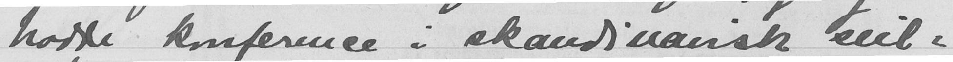hadde konference i skandinavisk seil-
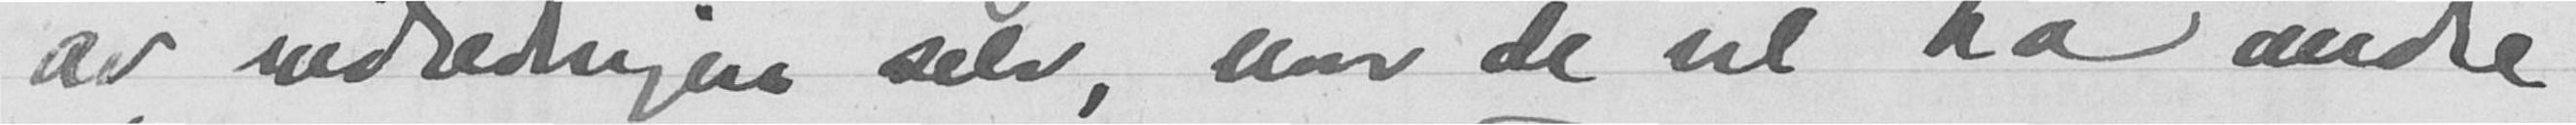av indredningen selv, naar de vil ha andre
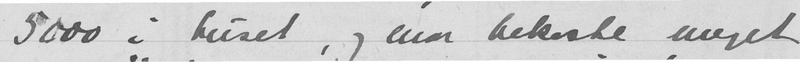5000 i huset, og maa bekoste meget
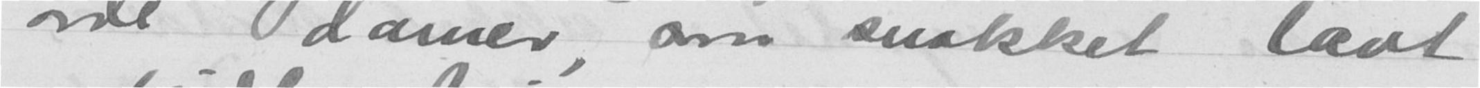øide damer, som snakket lavt
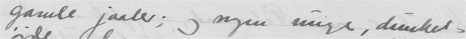gamle jaaler; og nogen unge, dunkel-
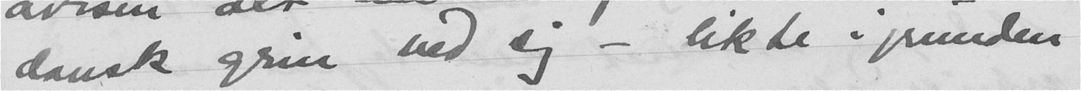dansk grin ved sig - likte i grunden
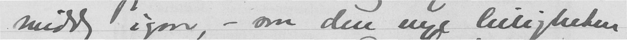middag igaar, - saa den nye leiligheten
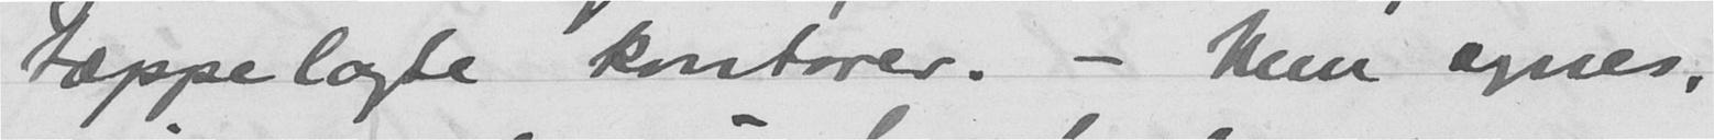tæppelagte kontorer. - Men synes,
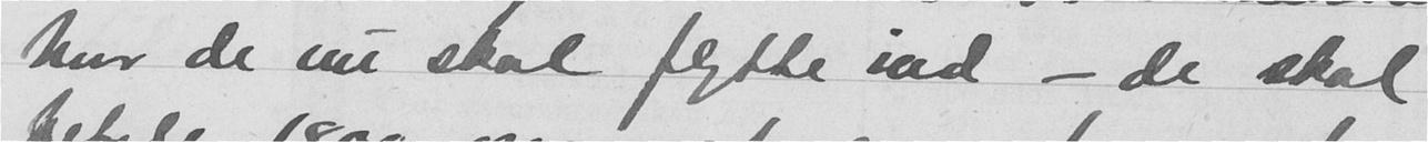hvor de nu skal flytte ind - de skal
